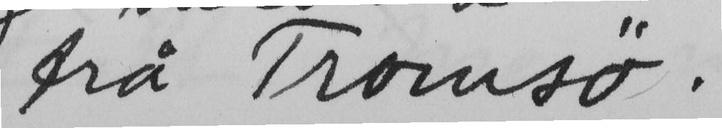frå Tromsö.
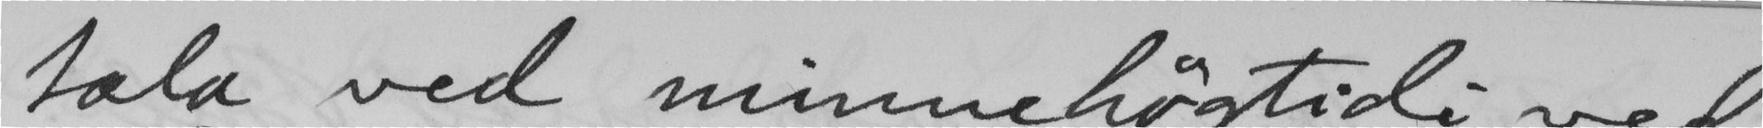tala ved minnehögtidi ved
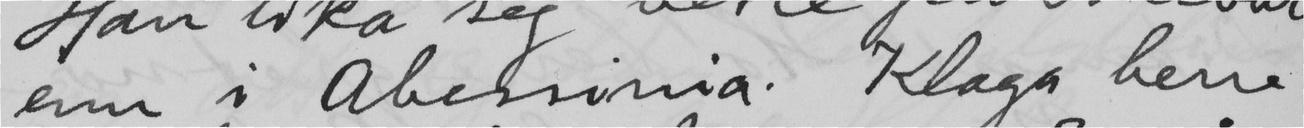enn i Abessinia. Klaga berre
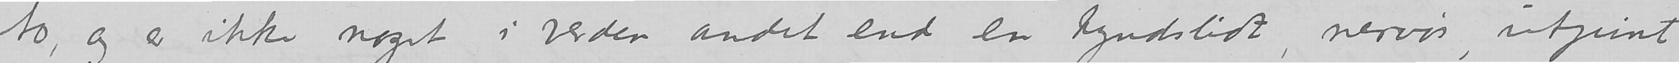to, og er ikke noget i verden andet end en tyndslidt, nervøs, utpint
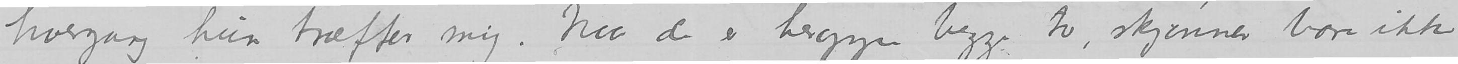hvergang hun træffer mig. Naar de er heroppe begge to, skjønner bare ikke
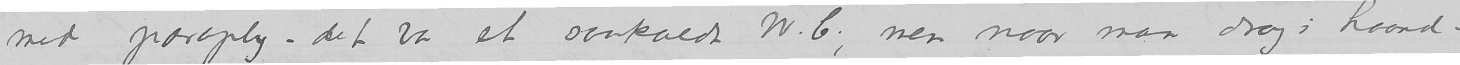med paraply – det var et saakaldt W.C., men naar man drog i haand-
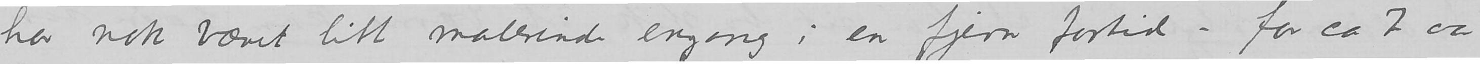har nok været litt malerinde engang i en fjern fortid – for ca 7 aar
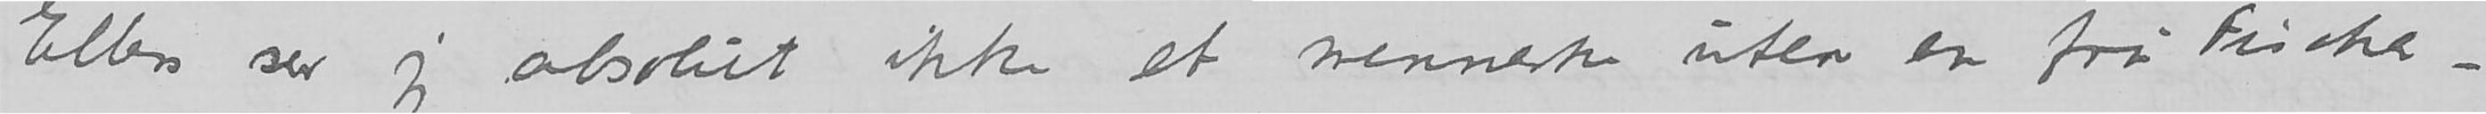Ellers ser jeg absolutt ikke et menneske uten en fru Fischer
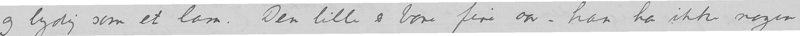og lydig som et lam. Den lille er bare fire aar – har har ikke nogen
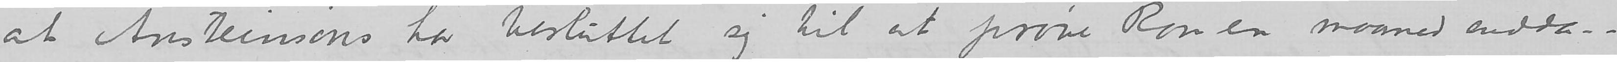at Ansteinsons har besluttet sig til at prøve Rom en maaned endda – –.
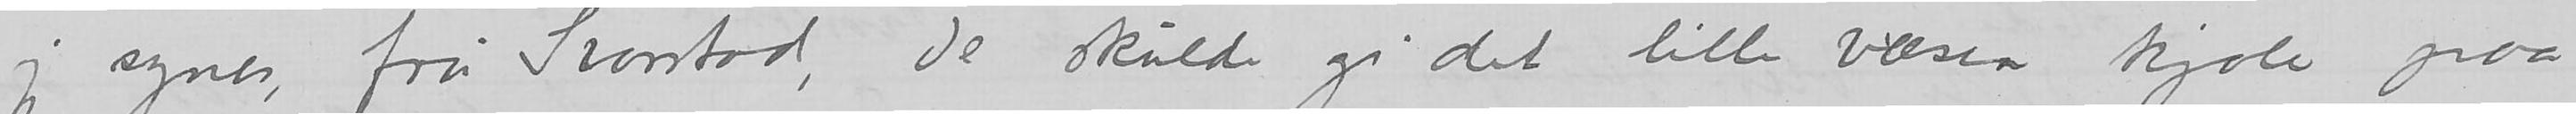«jeg synes, fru Svarstad, de skulde gi det lille væsen kjole paa
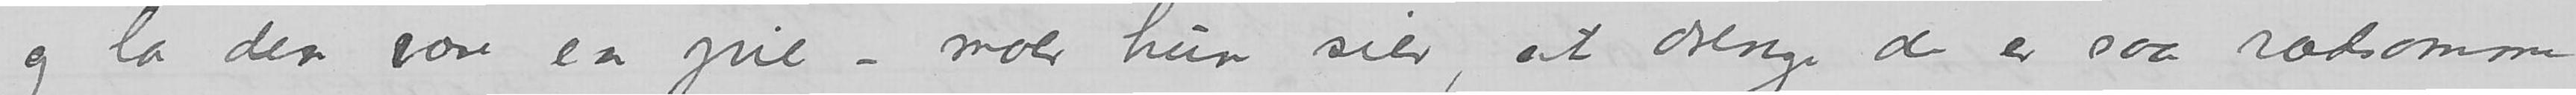og la den være en pie – moer hun sier, at drenger de er saa rædsomme»
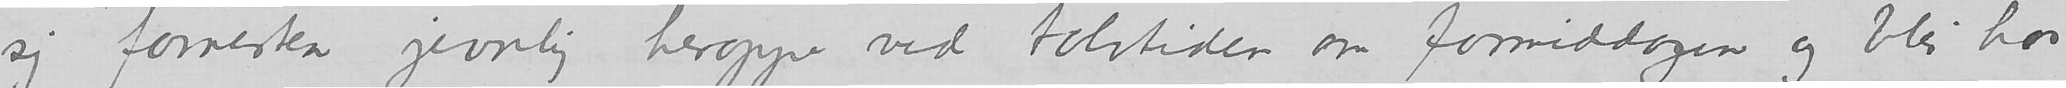sig forresten jevnlig heroppe ved tolvtiden om formiddagen og blir hos
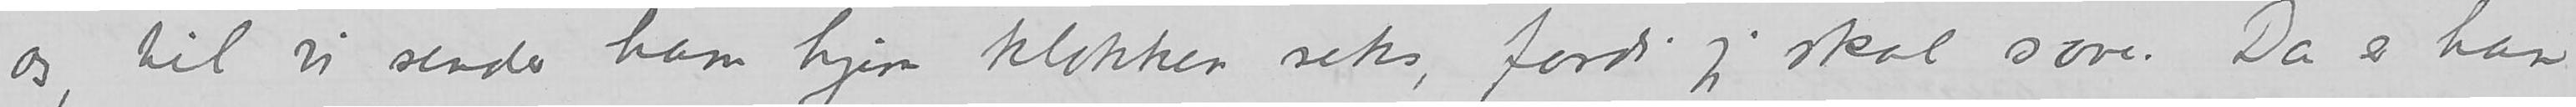os, til vi sender ham hjem klokken seks, fordi jeg skal sove. Da er han
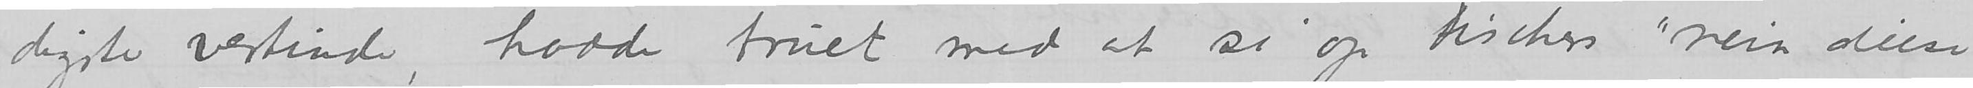modigste vertinde, hadde truet med at si op Fischers «nein diese
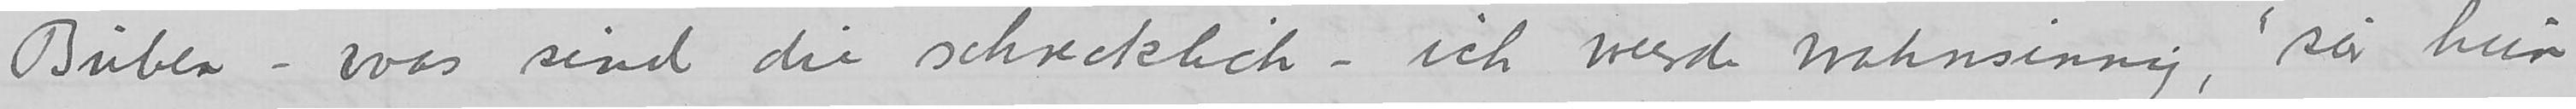Buben – was sind die schrecklich – ich werde wahnsinnig,» sier hun,
